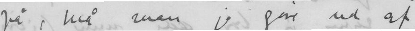på, må man jo gøre ud af
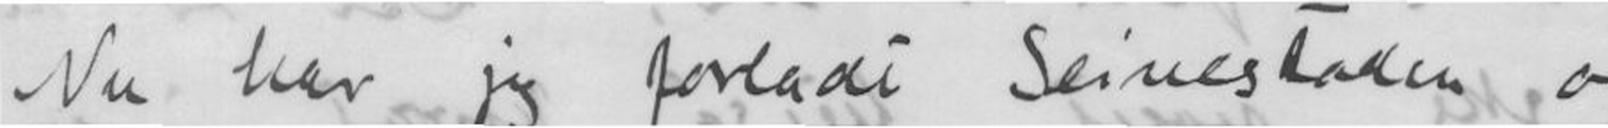Nu har jeg forladt Seinestaden, og
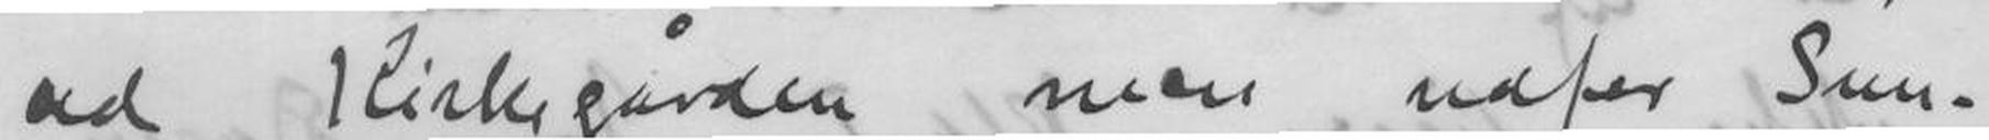ad Kirkegården men udfor Sun-
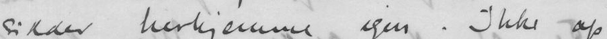sidder herhjemme igen. Ikke op
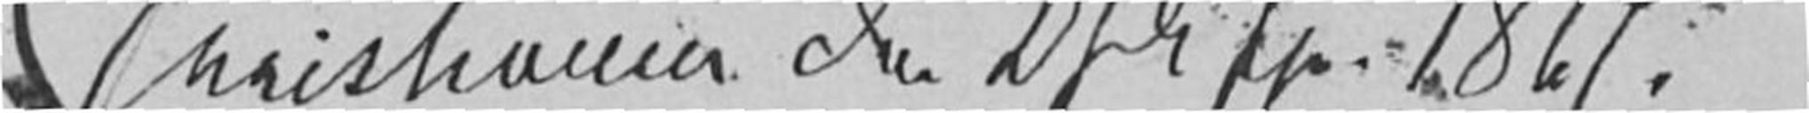Christiania den 27de Sp. 1867.
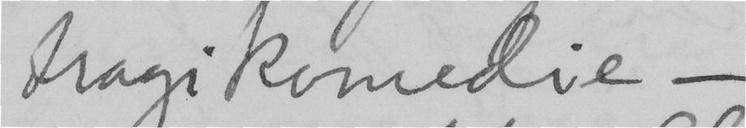tragikomedie –
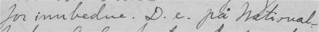for innbedne. D.e. på National-
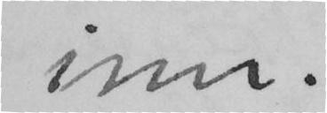inn.
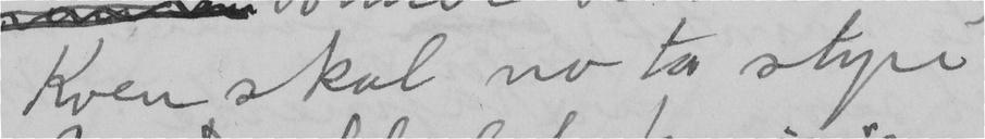Kven skal no ta styre
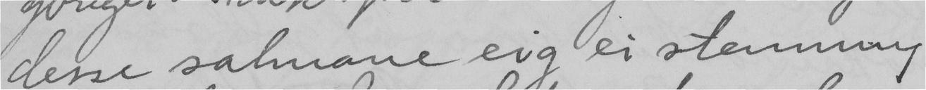desse salmane eig ei stemning
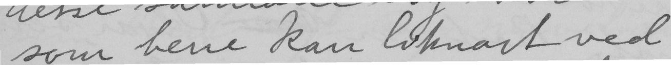som berre kan liknast ved
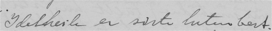I det heile er siste luten best.
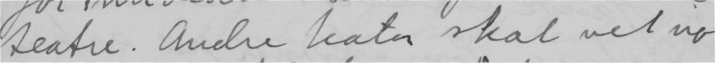teatre. Andre teater skal vel no
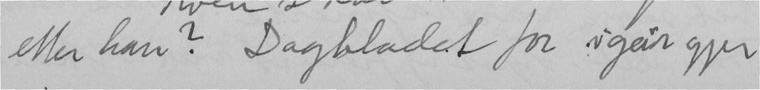etter han? Dagbladet for igår gjen
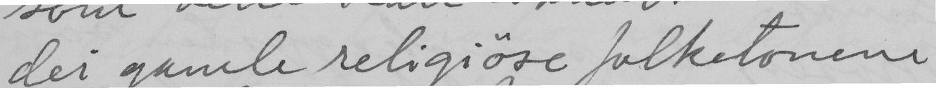der gamle religiöse folketonene
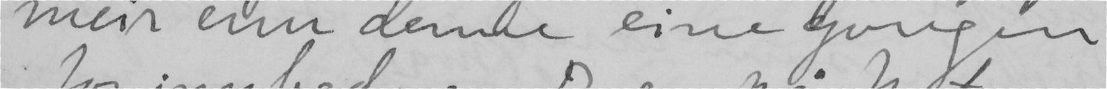meir enn denne eine gongen
